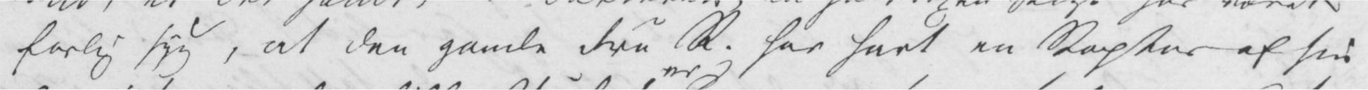farlig syg, at den gamle Fru R. har havt en Raptus af sin
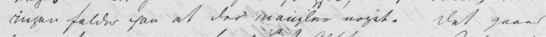ingen falder paa at der mangler noget. Det gaaer
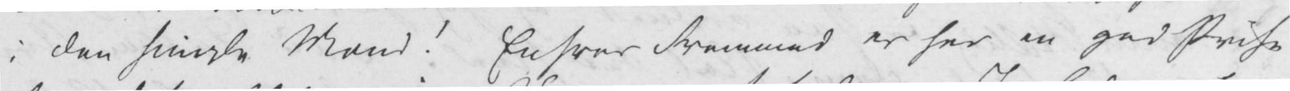i den simple Mand! Enhver Fremmed er her en god Prise
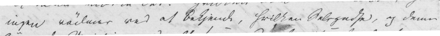ingen rødmer ved at bekjende, hvilken Selvgodhed, og denne
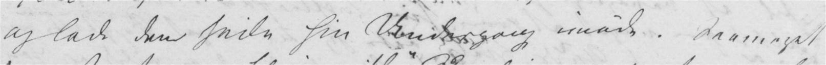og lade dem seile sin Undergang imøde. Saameget
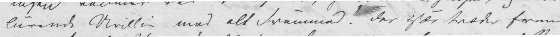lurende Uvillie mod alt Fremmed! der især træder frem
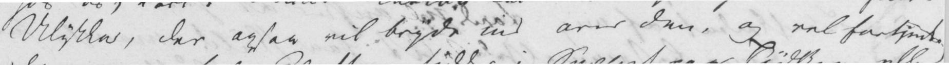Ulykker, der ogsaa vil bryde ind over den, og vel fortjent.
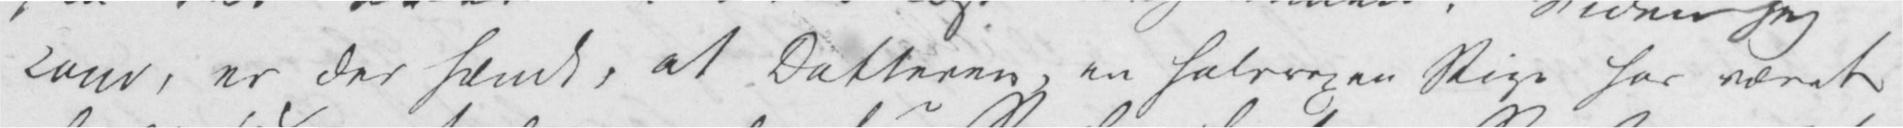kom, er der hændt, at Datteren, en halvvoxen Pige har været
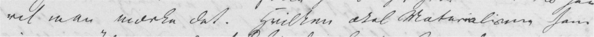vil man mærke det. Hvilken ækel Materialisme som
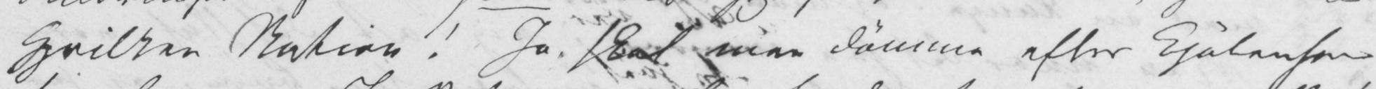Hvilken Nation! Ja, skal man dømme efter Kjøbenhavn
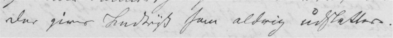Der gives Indtryk som aldrig udslettes.
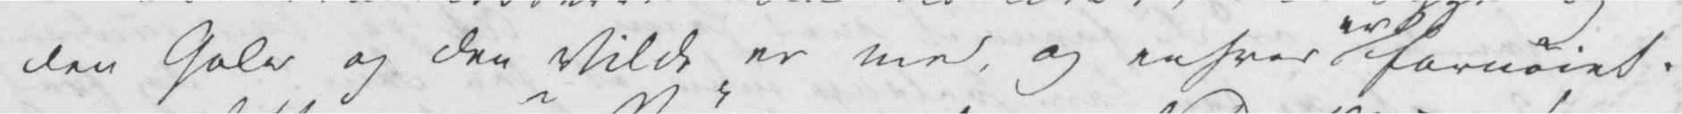den Gale og den Vilde er med, og enhver fornøiet.
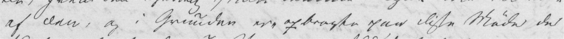af den, og i Grunden ere opbragte paa disse Møder der
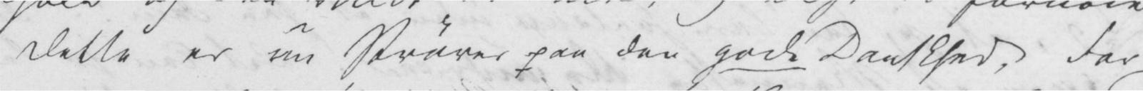Dette er nu Prøver paa den gode Danskhed. For
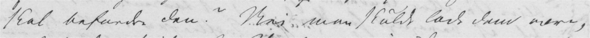skal befordre den? Nei man skulde lade dem være,
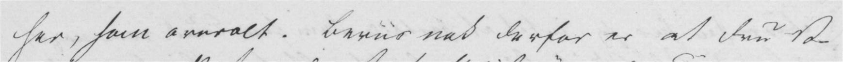her, som overalt. Beviis nok derfor er at Fru V.
